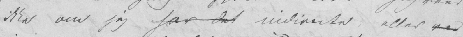ikke om jeg har det indirecte, eller ved
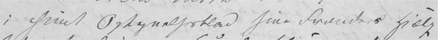i hiint Optegnelsesblad sine Frænders Hjælp
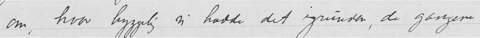om, hvor hyggelig vi hadde det igrunden, de gangene
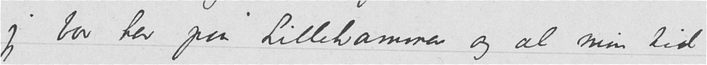- jeg bor her paa Lillehammer og al min tid
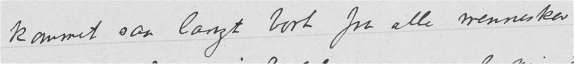kommet saa langt bort fra alle mennesker
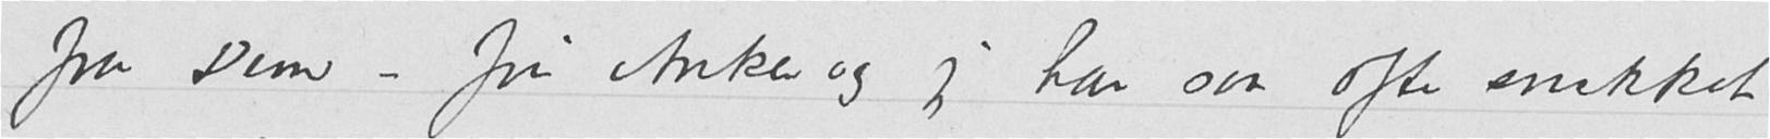fra Dem – fru Anker og jeg har saa ofte snakket
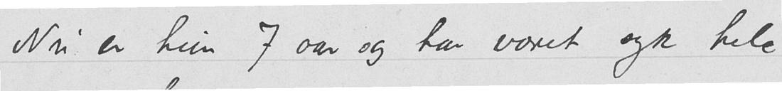Nu er hun 7 aar og har været syk hele
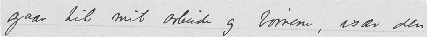gaar til mit arbeide og børnene, især den
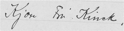Kjære Fru Kinck,
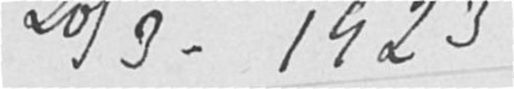20/3 - 1923
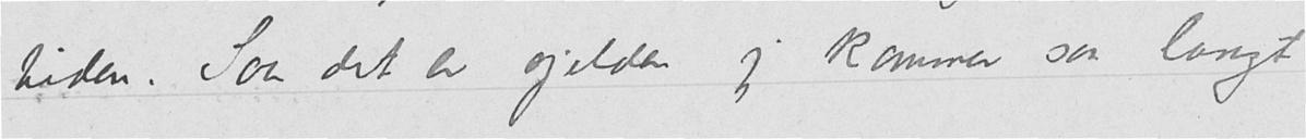tiden. Saa det er sjelden jeg kommer saa langt
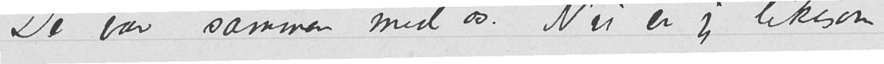De var sammen med os. Nu er jeg likesom
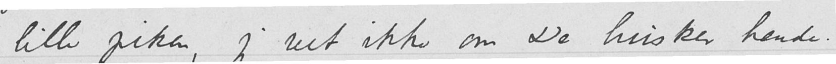lille piken, jeg vet ikke om De husker hende.
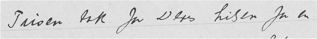Tusen tak for Deres hilsen for en
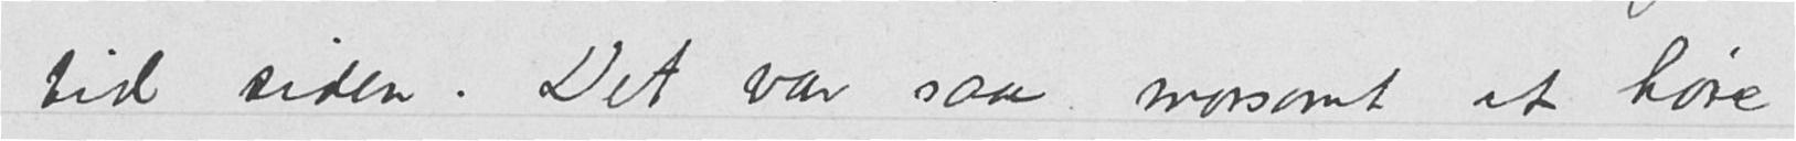tid siden. Det var saa morsomt at høre
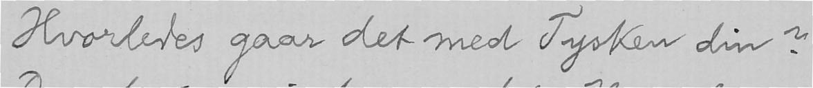Hvorledes gaar det med Tysken din?
